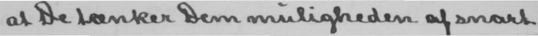at De tænker Dem muligheden af snart
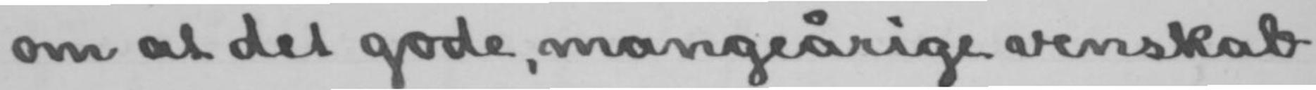om at det gode, mangeårige venskab
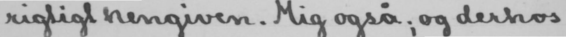rigtigt hengiven. Mig også; og derhos
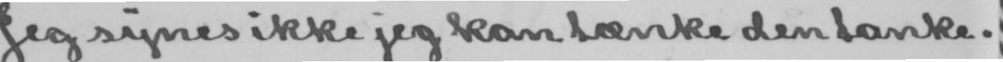Jeg synes ikke jeg kan tænke den tanke.
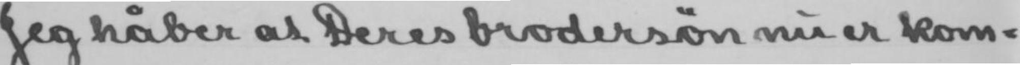Jeg håber at Deres brodersøn nu er kom-
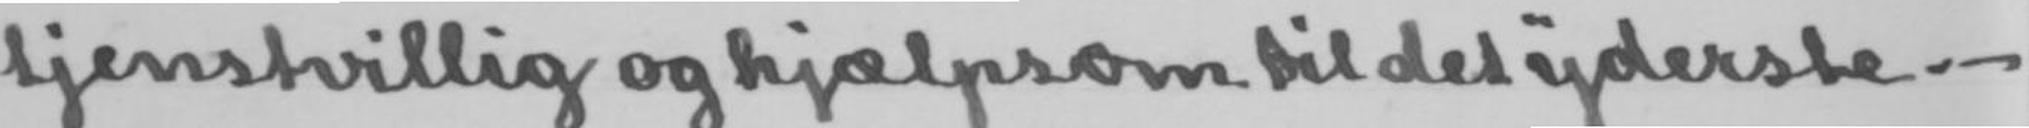tjenstvillig og hjælpsom til det yderste.-
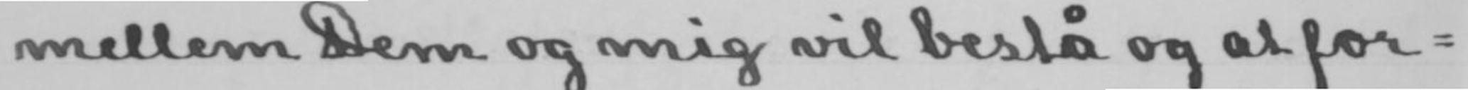mellem Dem og mig vil bestå og at for-
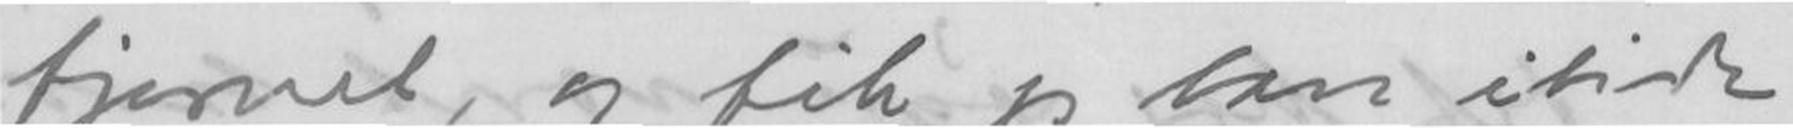fjernet, og fik jeg kun itide
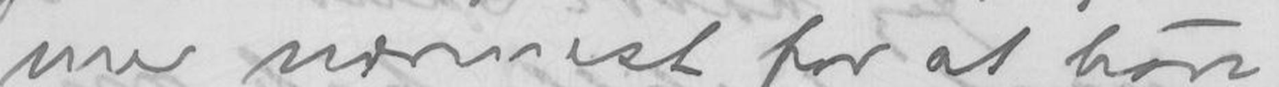mer nærmest for at høre,
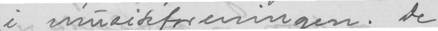imusikforeningen. De
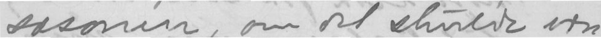sesonen, om det skulde være
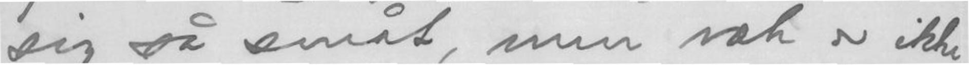sig så småt, men væk er ikke
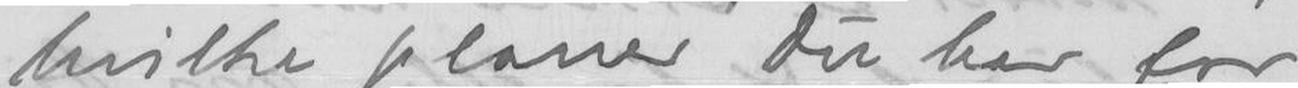hvilke planer du har for
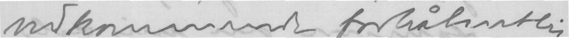vedkommende forhåbentlig
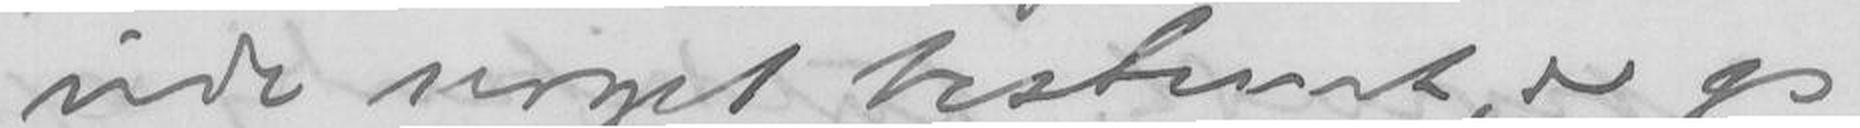vide noget bestemt, er jeg
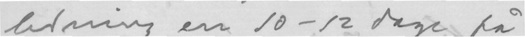ledning en 10-12 dage på
